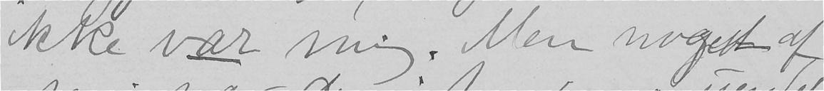ikke var mig. Men noget af
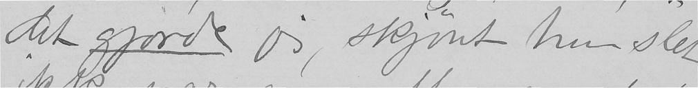det gjorde jeg, skjønt hun slet
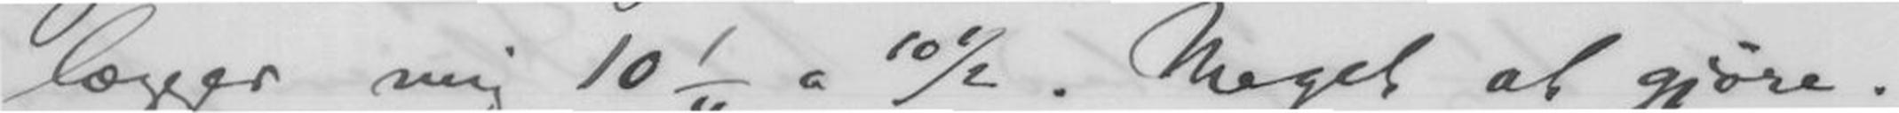lægger mig 10¼ a 10½. Meget at gjøre.
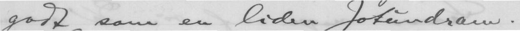godt som en liden Jotundram.
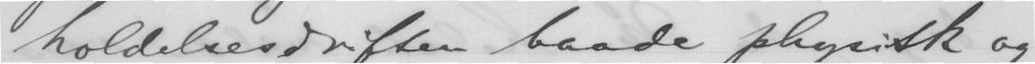holdelsesdriften baade physisk og
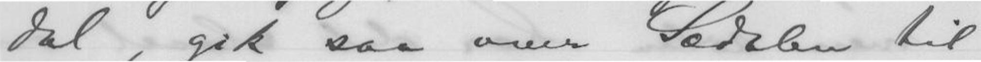dal, gik saa over Sædalen til
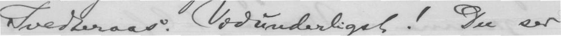Tvedteraas. Vidunderligst! Du ser
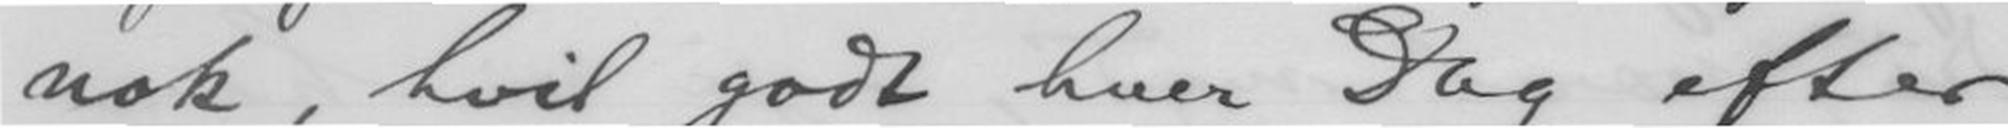nok, hvil godt hver Dag efter
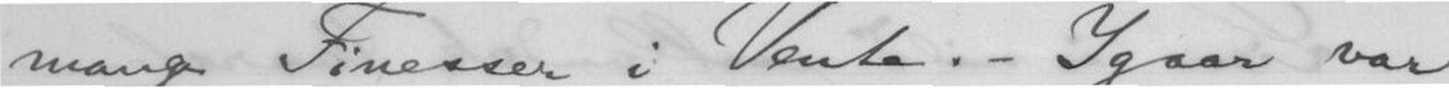mange Finesser i Vente. - Igaar var
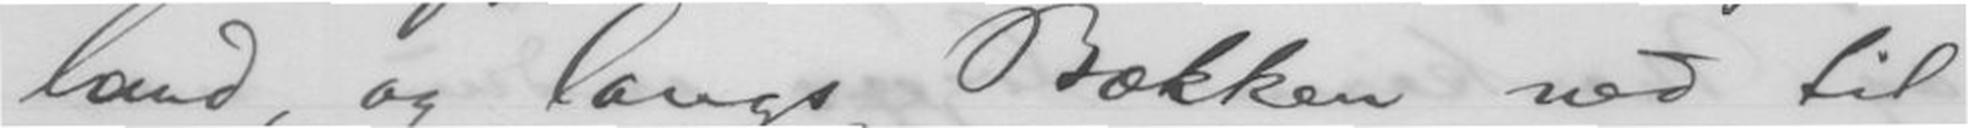land, og langs Bækken ned til
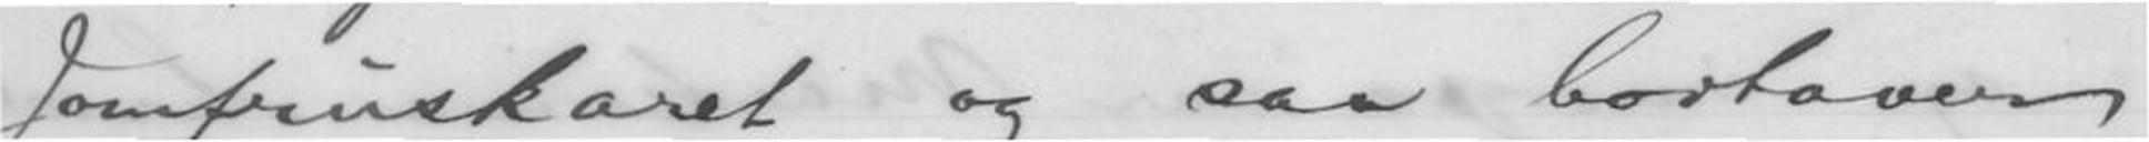Jomfruskaret og saa bortover
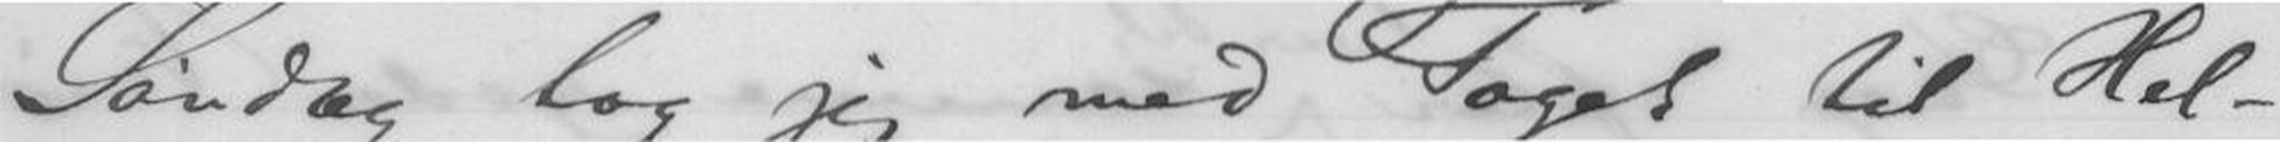Søndag tog jeg med Toget til Hel-
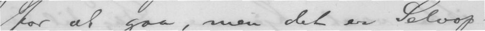for at gaa, men det er Selvop-
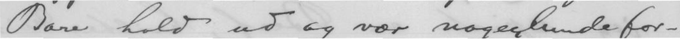Bare hold ud og vær nogenlunde for-
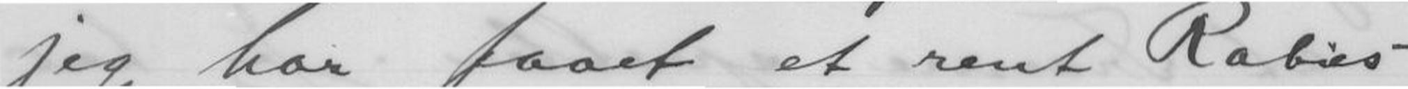jeg har faaet et rent Rabies
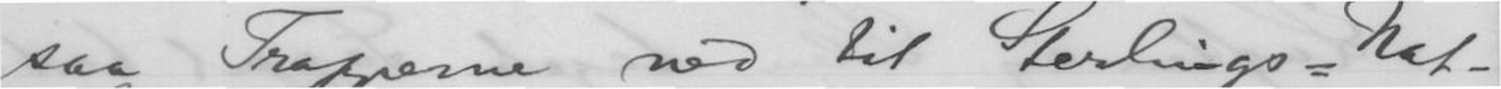saa Trapperne ned til Sterlings=Nat-
